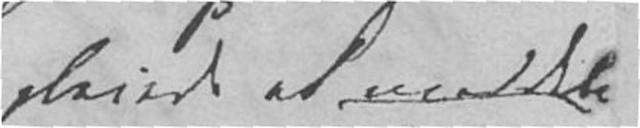pleiede at
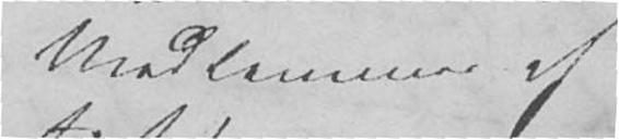Medlemmer af
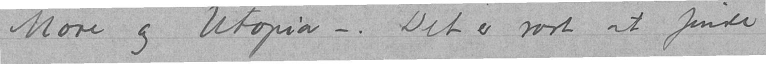More og Utopia –. Det er rart at finde
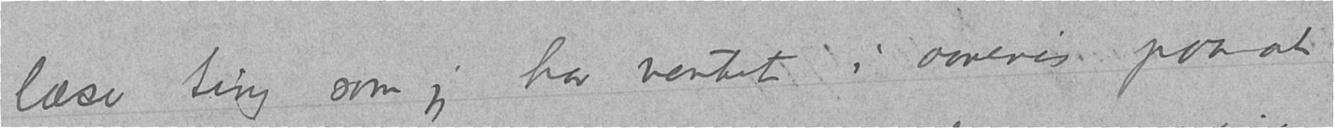læse ting som jeg har ventet i aarevis paa at
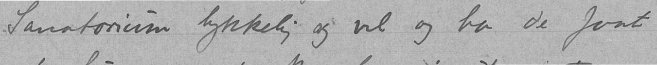Sanatorium lykkelig og vel og har de faat
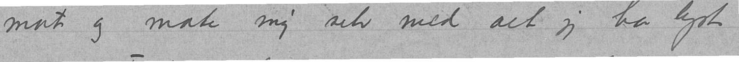mat og mate mig selv med alt jeg har lyst
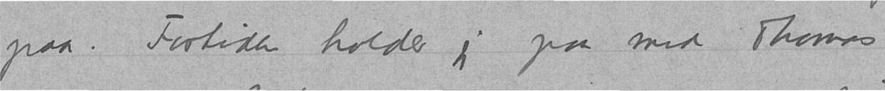paa. Fortiden holder jeg paa med Thomas
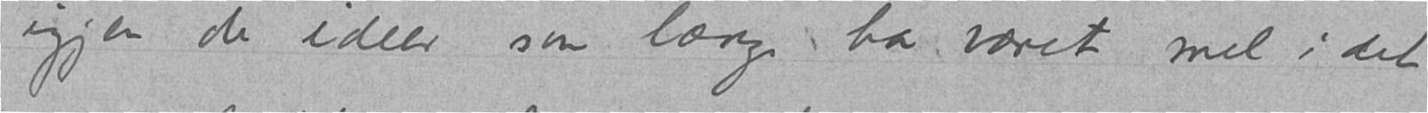igjen de ideer som længe har været med i det
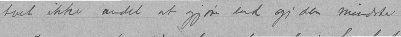talt ikke andet at gjøre end gi den mindste
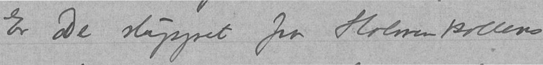Er De sluppet fra Holmenkollens
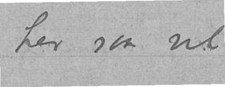Lev saa vel
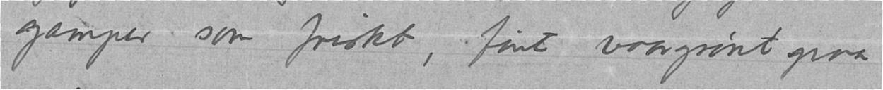gamper som friskt, fint vaargrønt paa
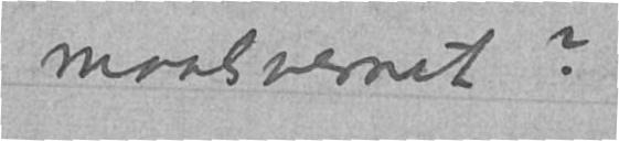maalsvernet ?
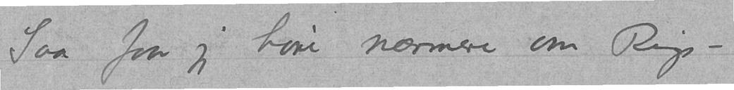Saa faar jeg høre nærmere om Rigs-
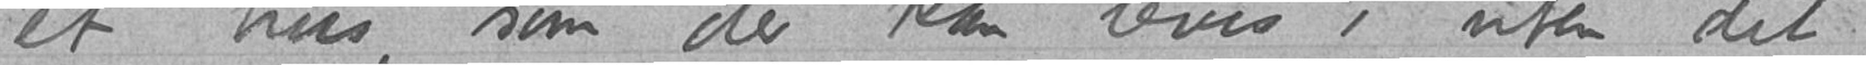et hus, som der kan leves i uten det
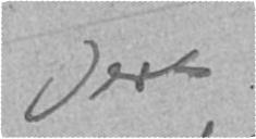Deres
